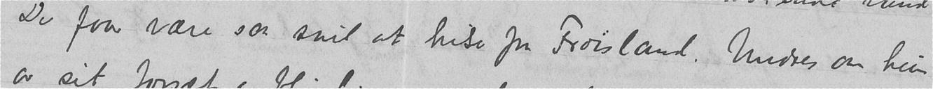De faar være saa snil at hilse fru Frøisland. Undres om hun
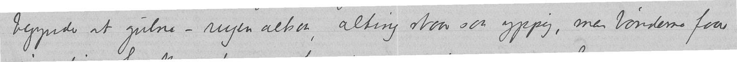begynder at gulne – rugen altsaa, alting staar saa yppig, men bønderne faar
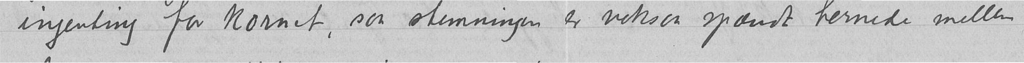ingenting for kornet, saa stemningen er noksaa spændt hernede mellem
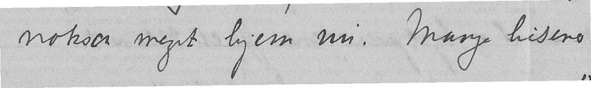noksaa meget hjem nu. Mange hilsener
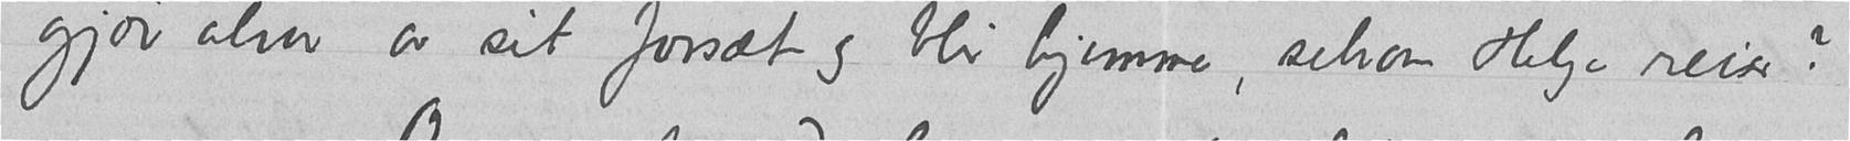gjør alvor av sit forsæt og blir hjemme, selvom Helge reiser?
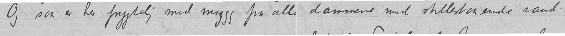Og saa er her frygtelig med mygg fra alle dammene med stillestaaende vand.
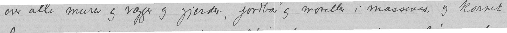over alle murer og vægger og gjerder, jordbær og moreller i massevis, og kornet
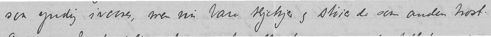saa yndig ivaares, men nu bare kjekjer og støier de som anden trost.
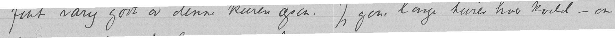faat varig godt av denne kuren ogsaa. Jeg gaar lange turer hver kveld – om
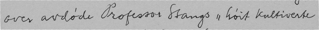over avdøde Professor Stangs "høit kultiverte
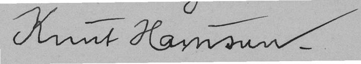Knut Hamsun.
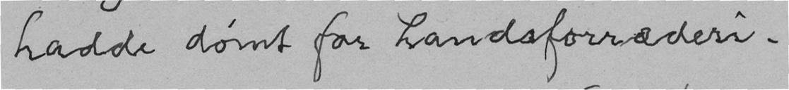hadde dømt for Landsforræderi.
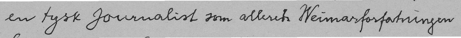en tysk Journalist som allerede Weimarforfatningen
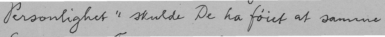Personlighet" skulde De ha føiet at samme
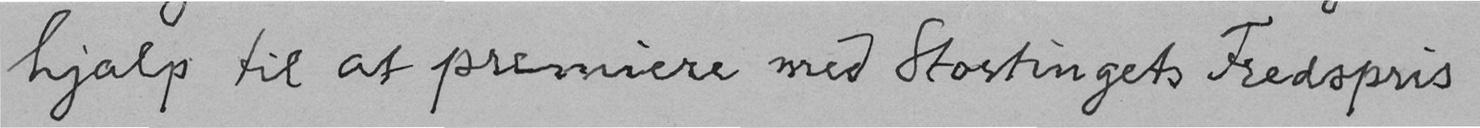hjalp til at premiere med Stortingets Fredspris
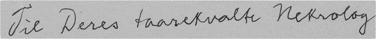Til Deres taarekvalte Nekrolog
